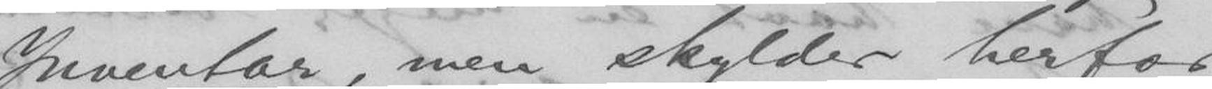Inventar, men skylder herfor
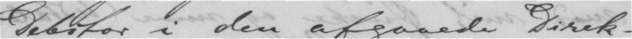Debitor i den afgaaede Direk-
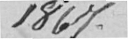1867.
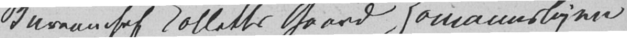Bureauchef Colletts Gaard Homannsbyen
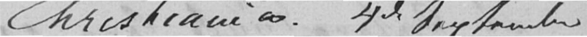Christiania. 4de September
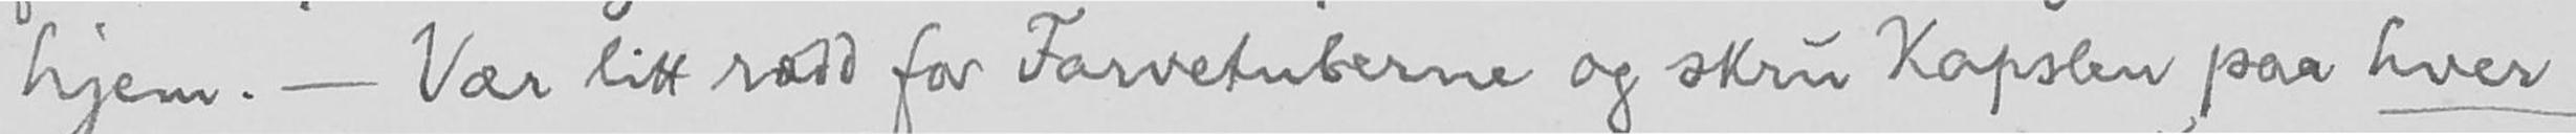hjem. - Vær litt rædd for Farvetuberne og skru Kapslen paa hver
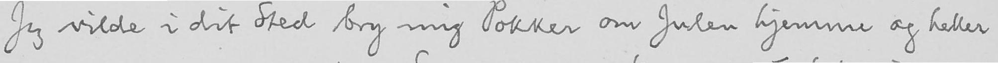Jeg vilde i dit Sted bry mig Pokker om Julen hjemme og heller
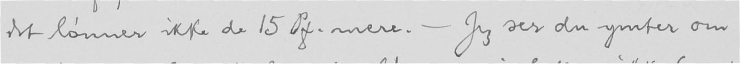det lønner ikke de 15 Pf. mere. - Jeg ser du ymter om
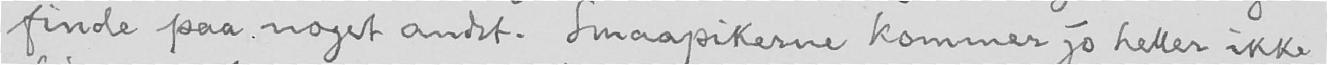finde paa noget andet. Smaapikerne kommer jo heller ikke
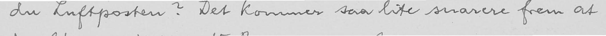du Luftposten? Det kommer saa lite snarere frem at
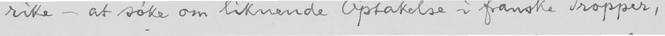rike - at søke om liknende Optakelse i franske Tropper,
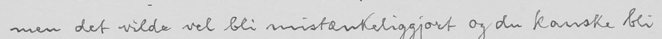men det vilde vel bli mistænkeliggjort og du kanske bli
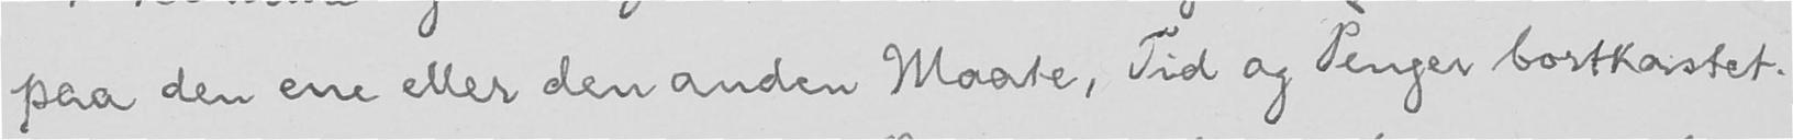paa den ene eller den anden Maate, Tid og Penger bortkastet.
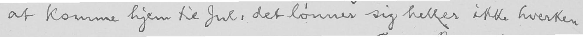at komme hjem til Jul, det lønner sig heller ikke hverken
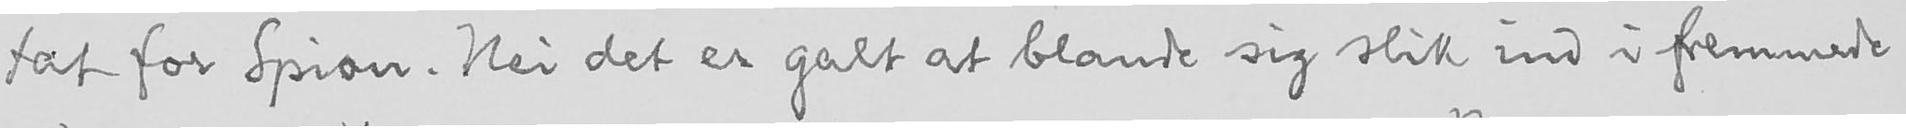tat for Spion. Nei det er galt at blande sig slik ind i fremmede
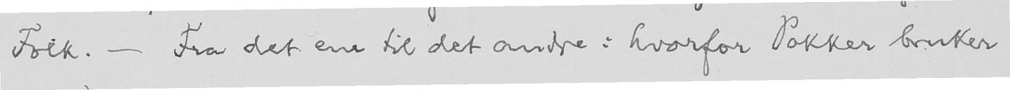Folk. - Fra det ene til det andre: hvorfor Pokker bruker
